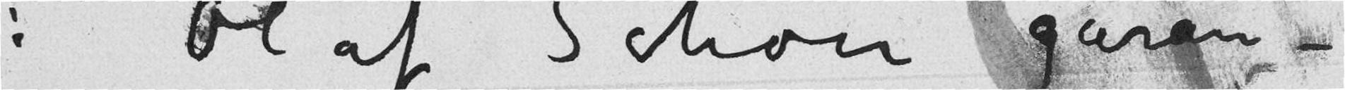: Olaf Schou garan-
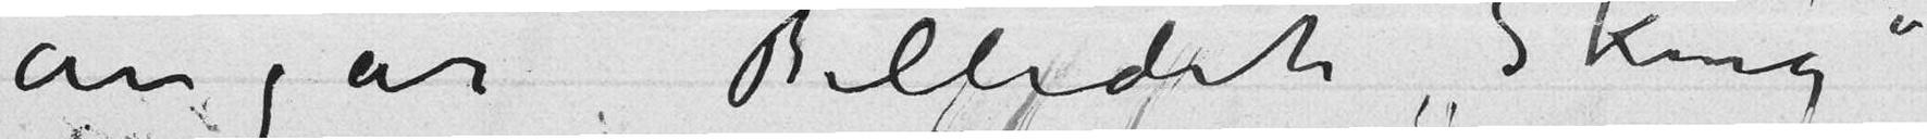angår Billedet «Skrig»
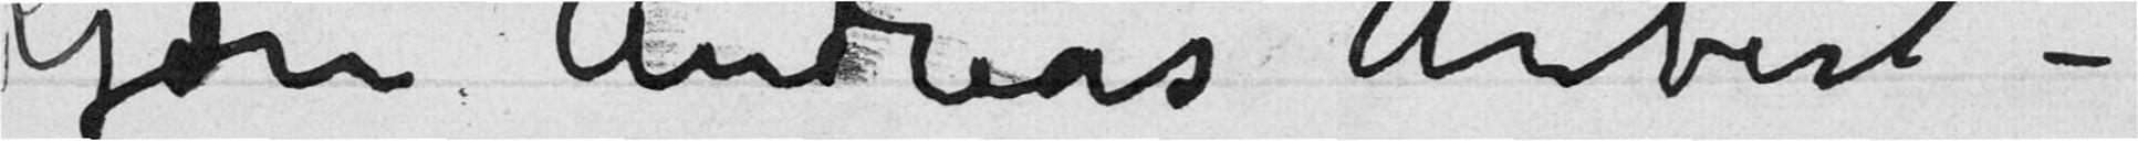Kjære Andreas Aubert –
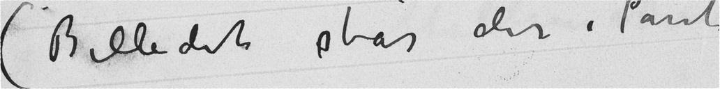(Billedet står der i Pant
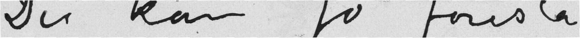De kan jo foreslå
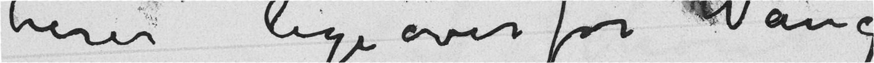terer ligeoverfor Wang
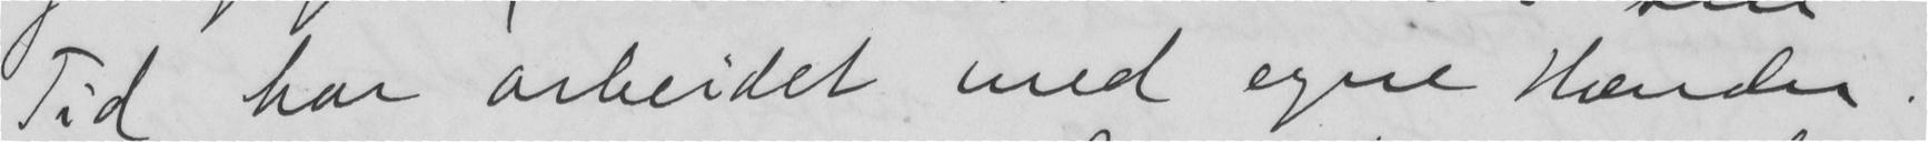Tid har arbeidet med egne Hænder.
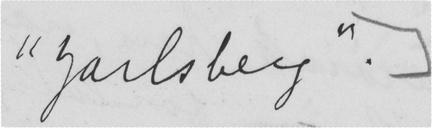"Jarlsberg."
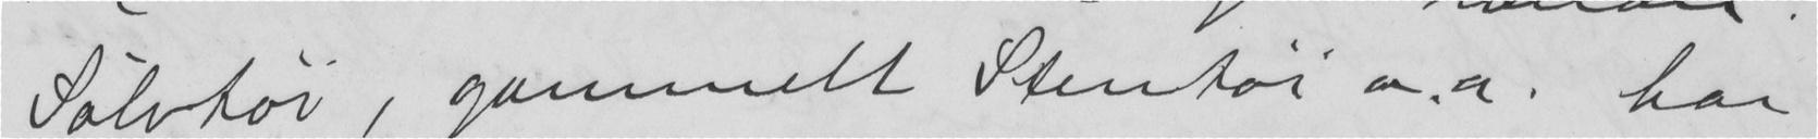Sølvtøi, gammelt Stentøi o.a. har
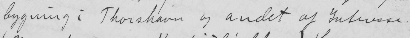bygning i Thorshavn og andet at Interesse.
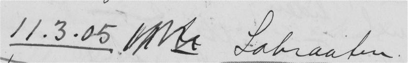11.3.05  Labraaten.
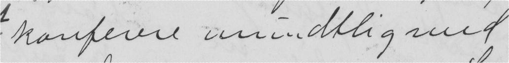konferere mundtlig med
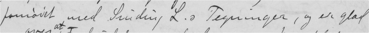fornøiet med Sinding L. s Tegninger, og er glad
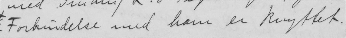Forbindelse med ham er knyttet.
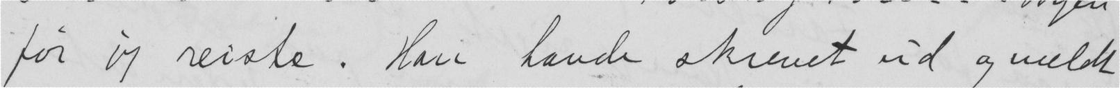før jeg reiste. Han havde skrevet ud og meldt
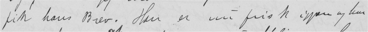fik hans Brev. Han er nu frisk igjen og har
